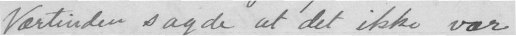Værtinden sagde at det ikke var
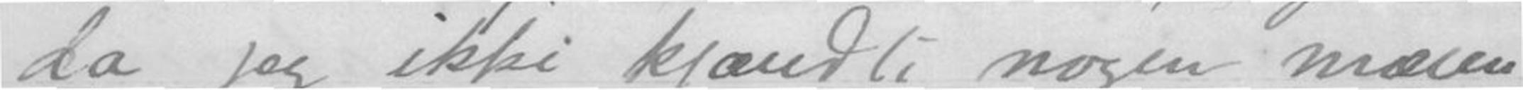da jeg ikke kjændte nogen mæçen
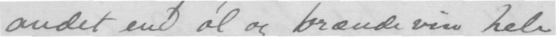andet end øl og brændevin hele
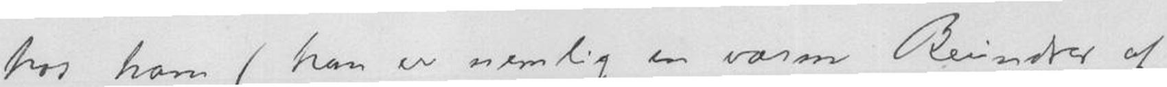hos ham (han er nemlig en varm Beundrer af
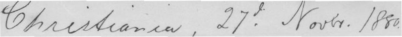Christiania 27 Novbr. 1880
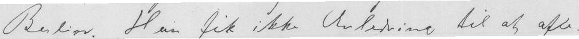Berlin. Han fik ikke Anledning til at afle-
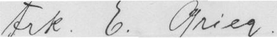Frk. E. Grieg
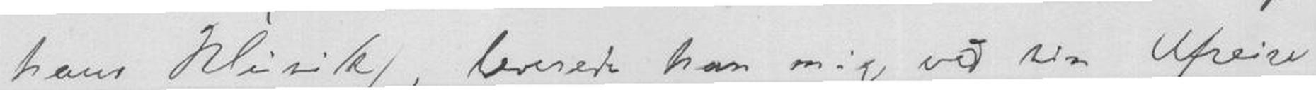hans Musik, leverede ham mig ved sin Afreise
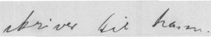skriver til ham
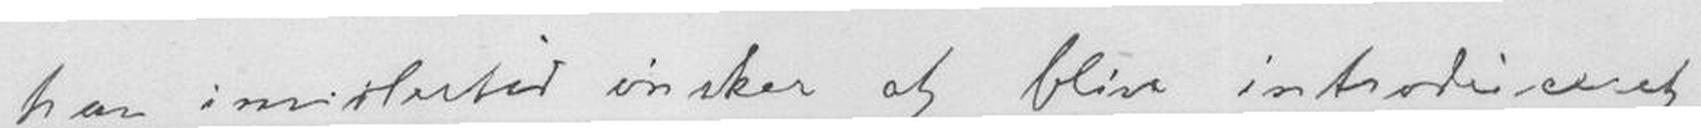han imidertid ønsker at blive introduseret
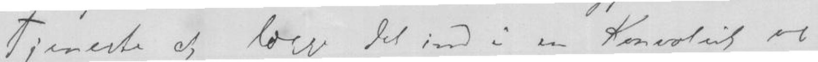tjeneste at lægge det ind i en Konvolut og
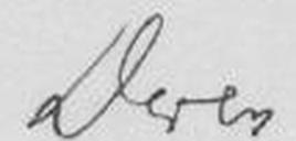Deres
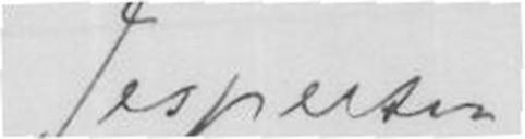J.Jespersen
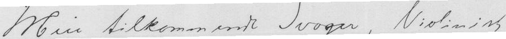Min tilkommende Svoger, Violinist
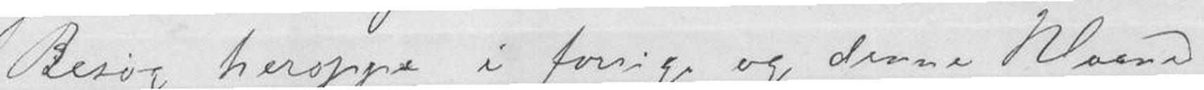Besøg heroppe i forrige og denne Maaned
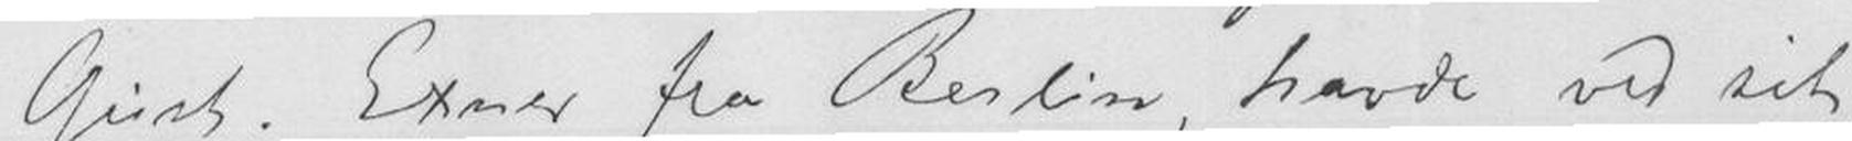Gust. Exner fra Berlin, havde ved sit
